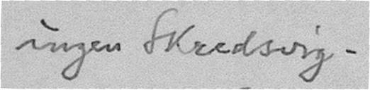ingen Skredsvig.
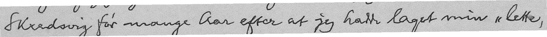Skredsvig før mange Aar efter at jeg hadde laget min "lette,
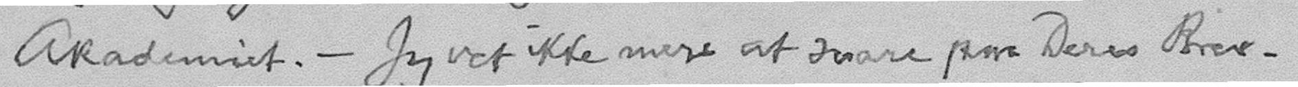Akademiet. - Jeg vet ikke mere at svare paa Deres Brev.
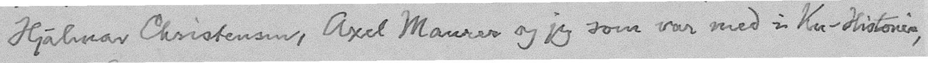Hjalmar Christensen, Axel Maurer og jeg som var med i Ku-Historien,
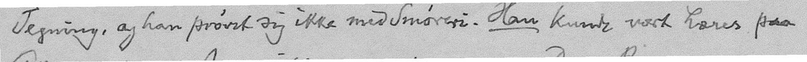Tegning, og han prøvet sig ikke med Smøreri. Han kunde vært Lærer paa
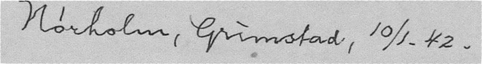Nørholm, Grimstad, 10/1. 42.
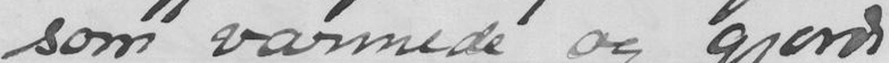som varmede og gjorde
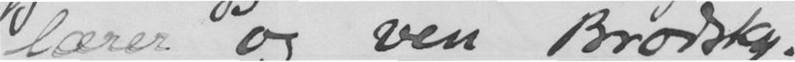lærer og ven Brodsky.
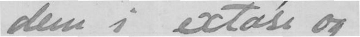dem i extase og
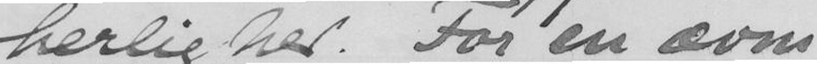herlighed. Foe en ævne
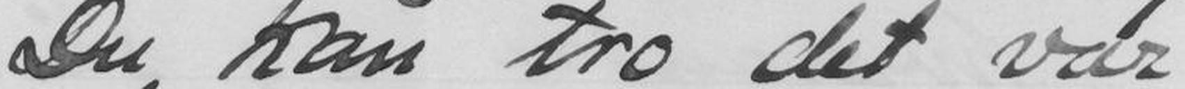Du kan tro det var
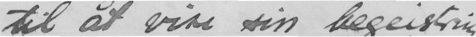til at vise sin begeistring
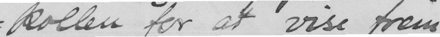kollen for at vise frem
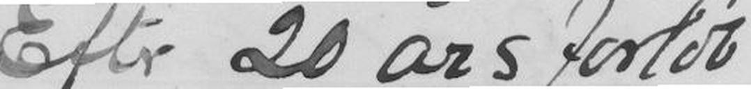Efter 20 års forløb
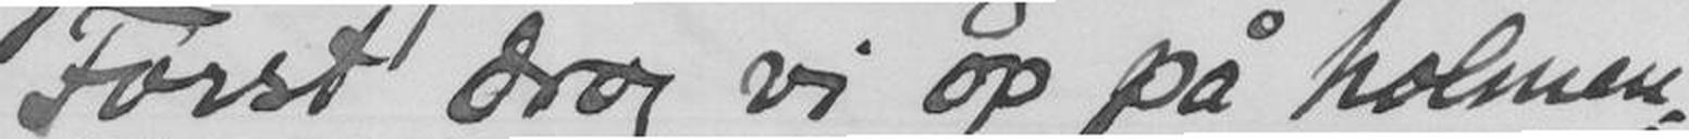Først drog vi op på holmen-
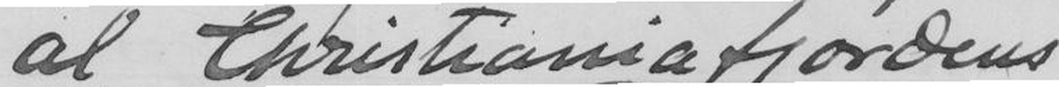al Christianiafjordens
